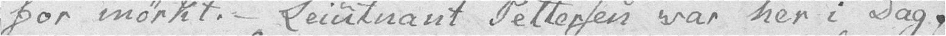for mørkt. – Leiutnant Pettersen var her i Dag.
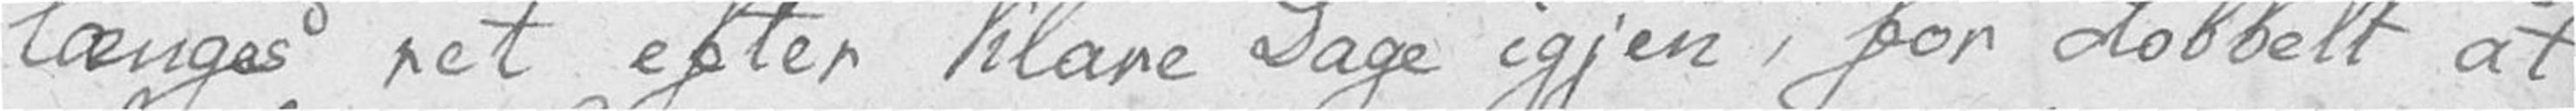længes ret efter klare Dage igjen, for dobbelt at
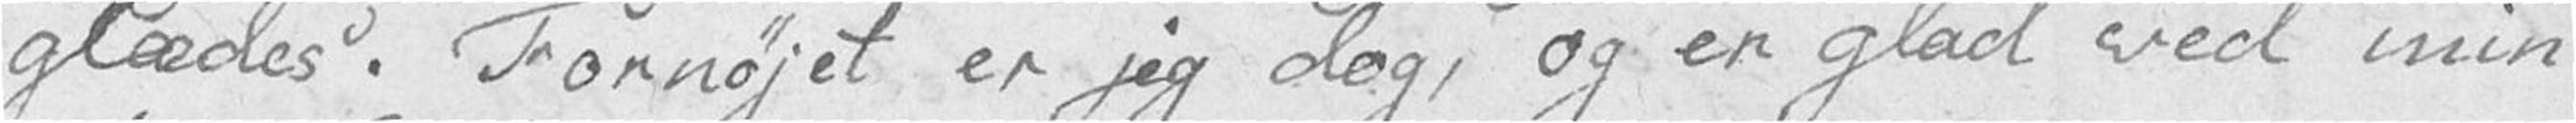glædes. Fornøjet er jeg dog, og er glad ved min
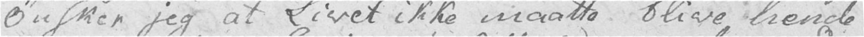Ønsker jeg at Livet ikke maatte blive hende
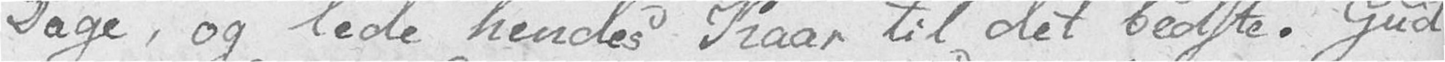Dage, og lede hendes Kaar til det bedste. Gud
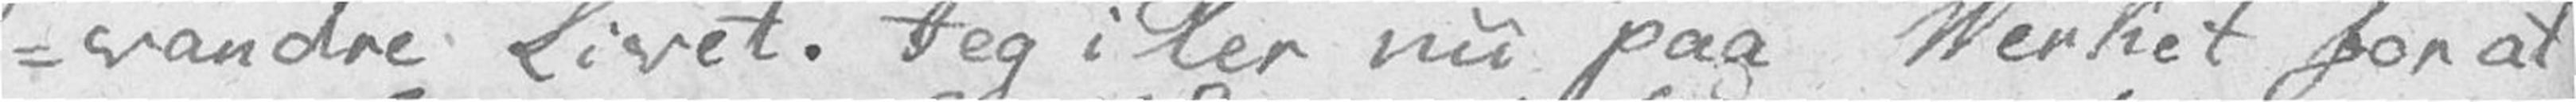-vandre Livet. Jeg iler nu paa Verket for at
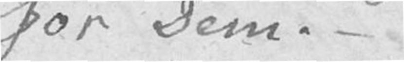for Dem. –
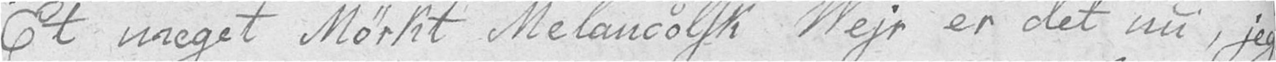Et meget Mørkt Melancolsk Vejr er det nu, jeg
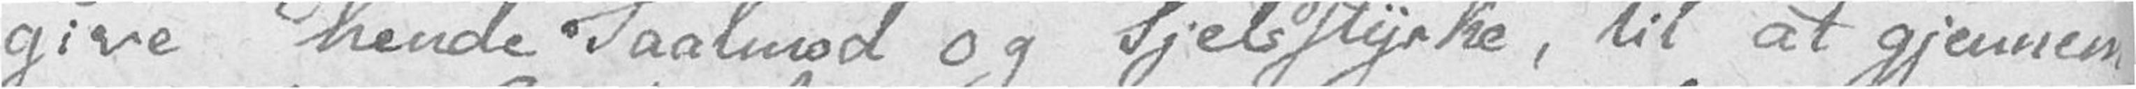give hende Taalmod og Sjelsstyrke, til at gjennem
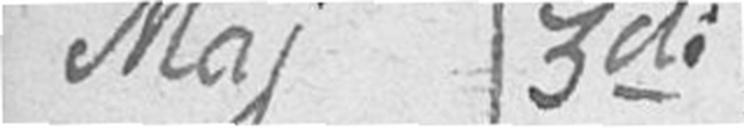Maj 3di
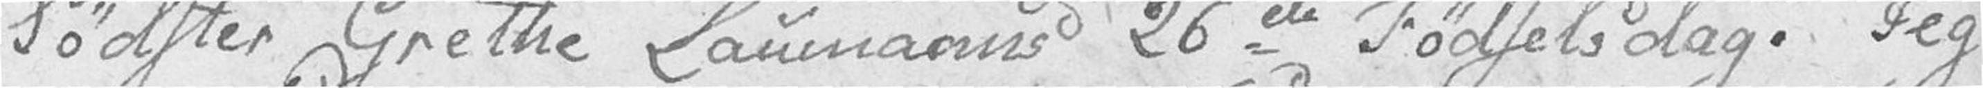Sødster Grethe Laumanns 26de Fødselsdag. Jeg
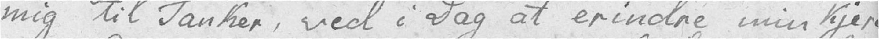mig til Tanker, ved i Dag at erindre min kjere
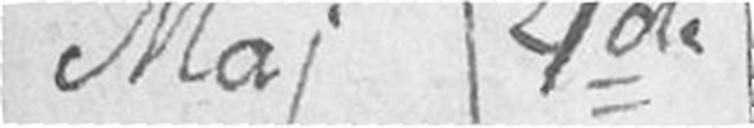Maj 4de
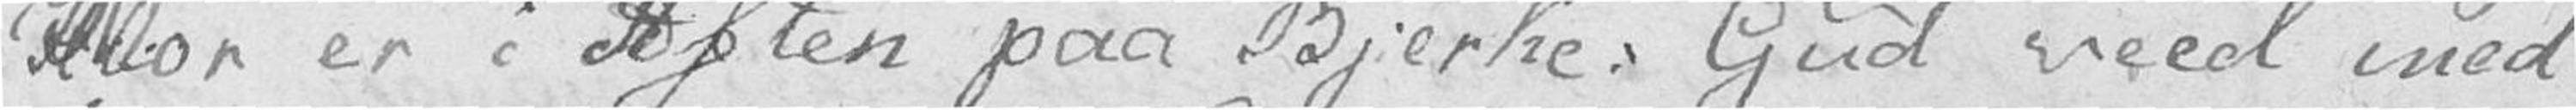Mor er i Aften paa Bjerke. Gud veed med
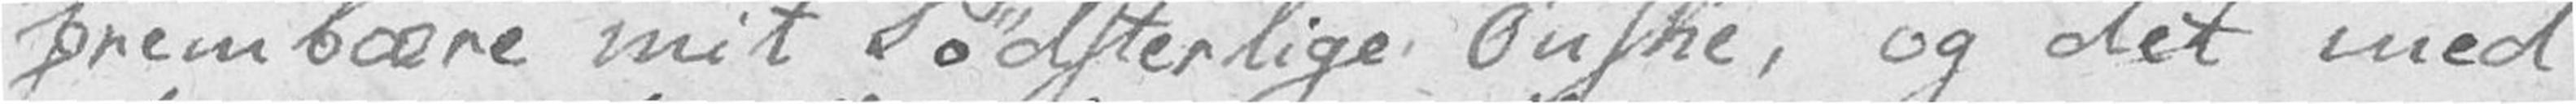frembære mit Sødsterlige Ønske, og det med
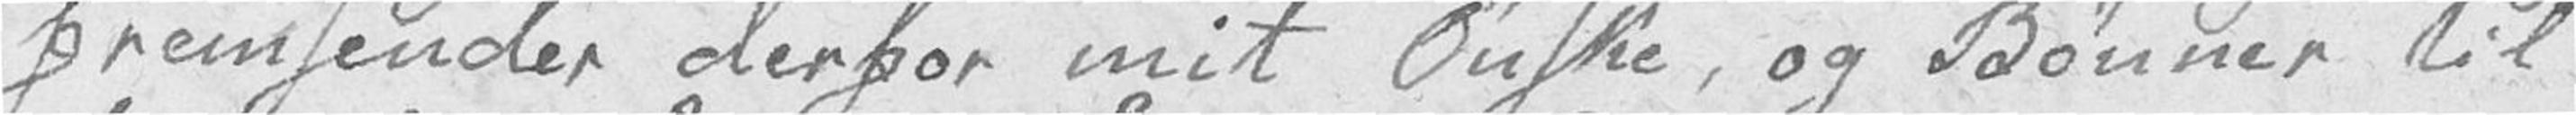fremsender derfor mit Ønske, og Bønner til
